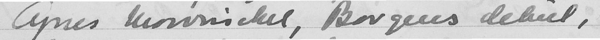Agnes Mowinckel, Borgens debut,
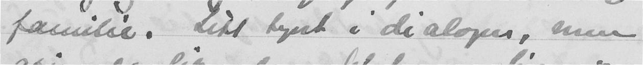familie. Litt tynt i dialogen, men
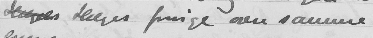Helges forrige over samme
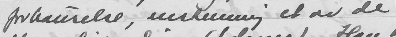forbauselse, enstemmig et av de
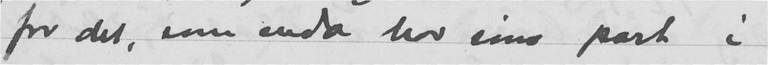for det, som enda har sin part i
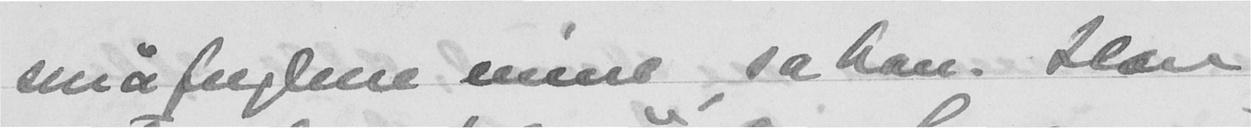småfuglene mine sa han. Han
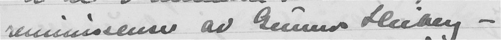reminissene av Gunnar Heiberg -
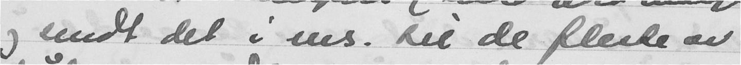og sendt det i ms. til de fleste av
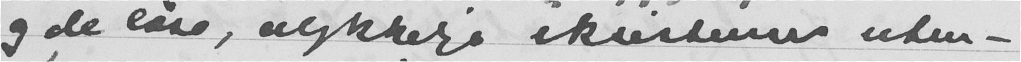og de løse, ulykkelige eksistenser uten-
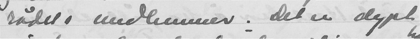rådets medlemmer. Det er dypt
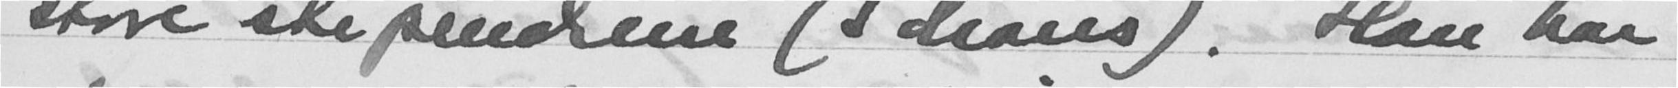store stipendiene (S deans). Han har
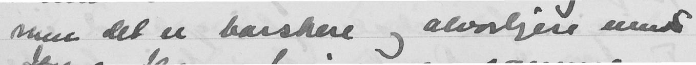men det er barskere og alvorligere enn
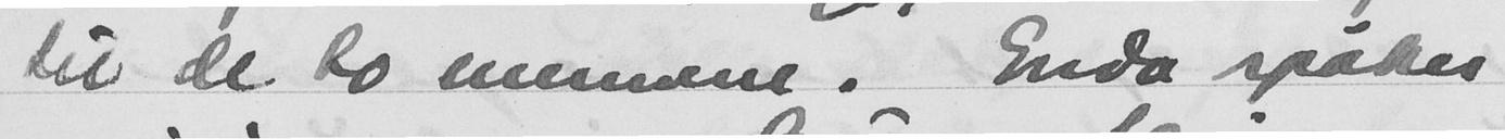til de to mennene. Enda spøker
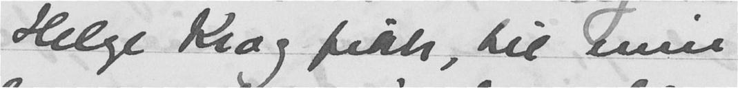Helge Krog fikk, til min
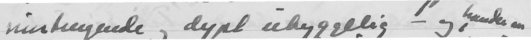inntrengende og dypt uhyggelig - og handler om
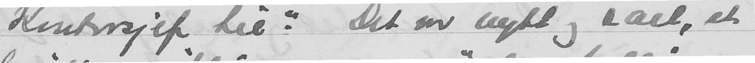"Kontorsjef Lie." Det var nytt og rart, et
